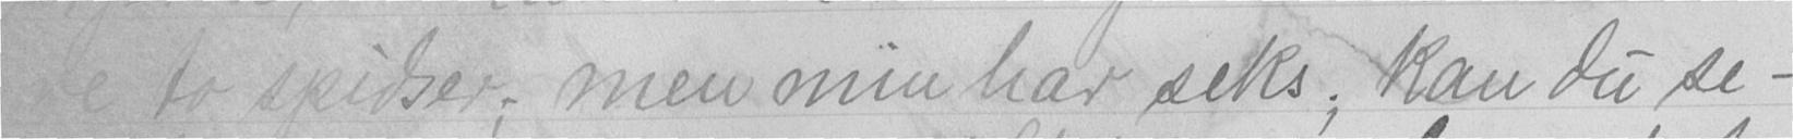re to spidser; men min har seks; kan du se-
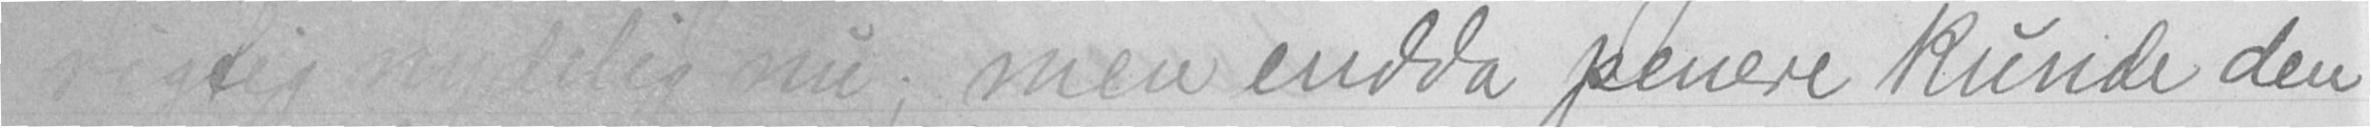rigtig nydelig nu; men endda penere kunde den
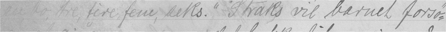en, to, tre, fire fem, seks." Straks vil barnet forsø-
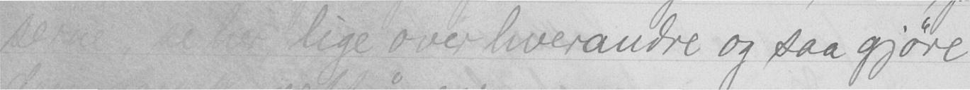serne, se her lige over hverandre og saa gjøre
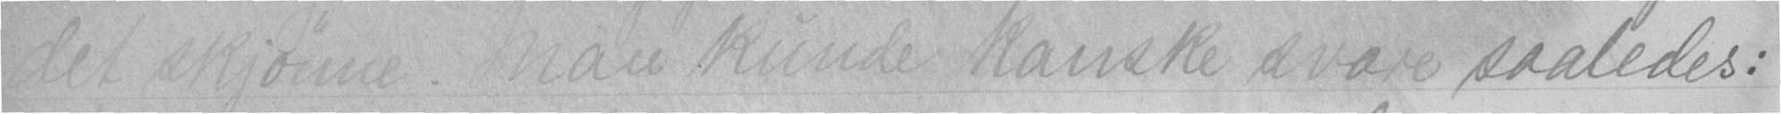det skjønne. Man kunde kanske svare saaledes:
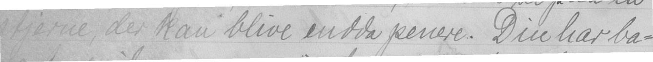stjerne, der kan blive endda penere. Din har ba-
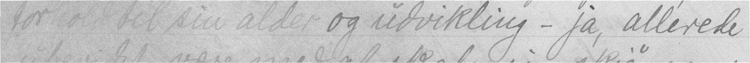forhold til sin alder og udvikling - ja, allerede
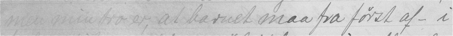men min tro er, at barnet maa fra først af - i
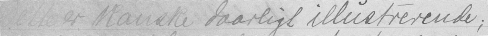Dette er kanske daarligt illustrerende;
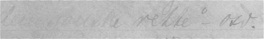dem ganske rette" - osv.
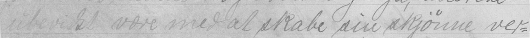ubevidst være med at skabe sin skjønne ver-
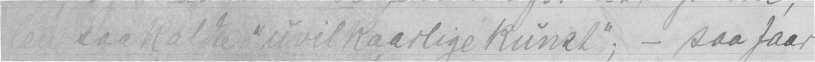den saakaldte "uvilkaarlige kunst"; - saa faar
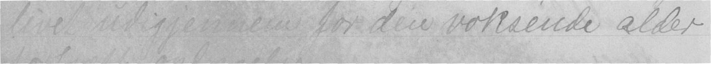livet udigjennem for den voksende alder
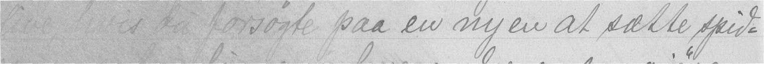blive, hvis du forsøgte paa en ny en at sætte spid-
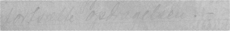fortsætte opfragelsen. -
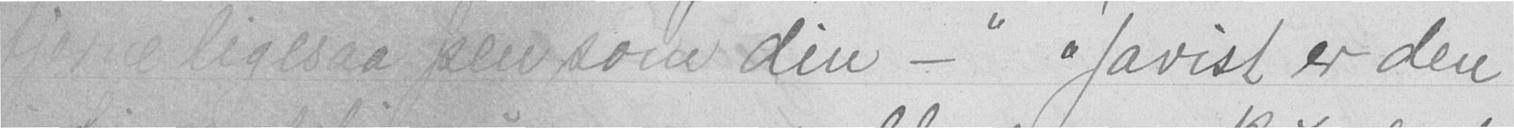stjerne ligesaa pen som din -" "Javist er den
